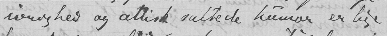ivrighed og attisk saltede humor er lige
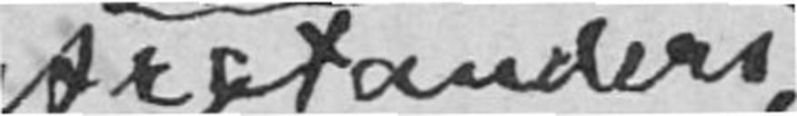Arctanders,
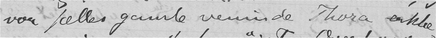vor fælles gamle veninde Thora erklæ-
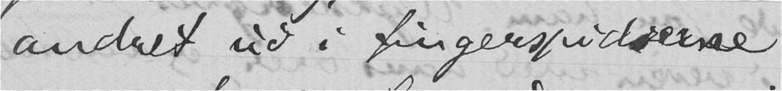andret ud i fingerspidserne.
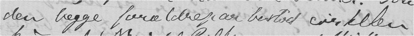den begge forældrepar bestod cirkelen
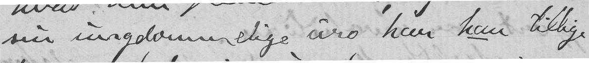sin ungdommelige uro har han tillige
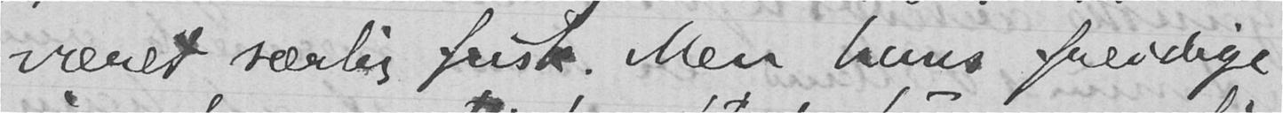været særlig frisk. Men hans freidige
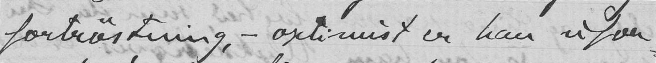fortrøstning, - optimist er han ufor-
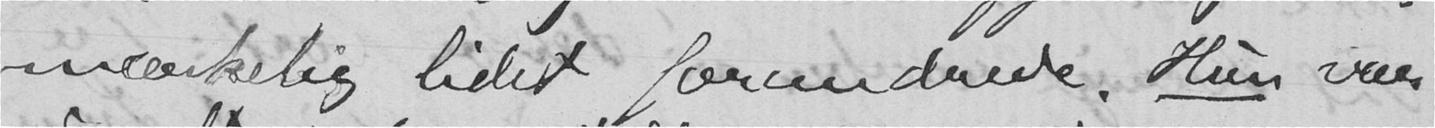mærkelig lidet forandrede. Hun var
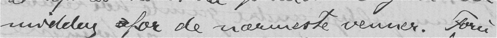middag for de nærmeste venner. Foru-
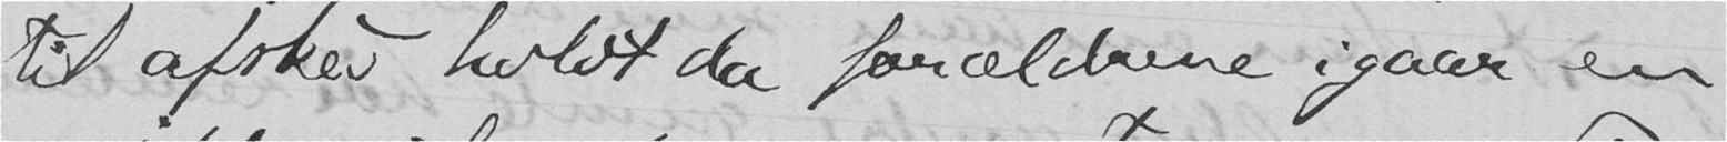til afsked holdt da forældrene igaar en
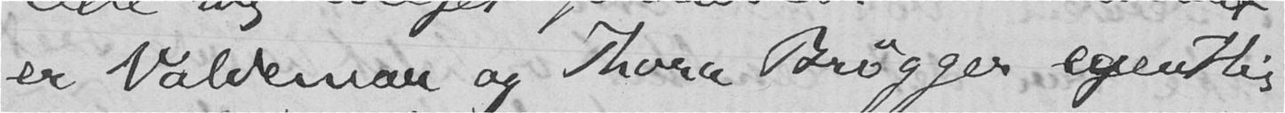er Valdemar og Thora Brøgger egentlig
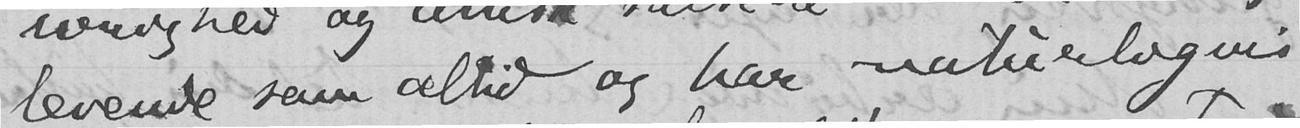levende som altid og har naturligvis
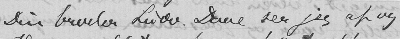Din broder Ludv. Daae ser jeg af og
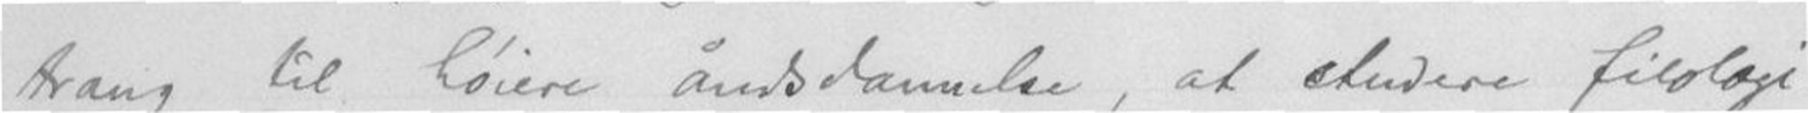trang til høiere åndsdannelse, at studere filologi
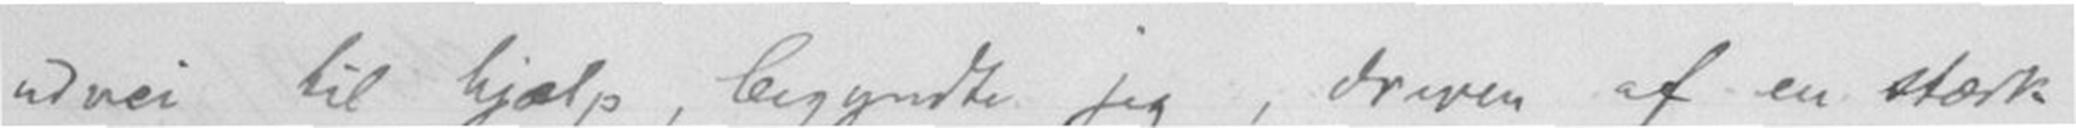udvei til hjælp, begyndte jeg, dreven af en stærk
